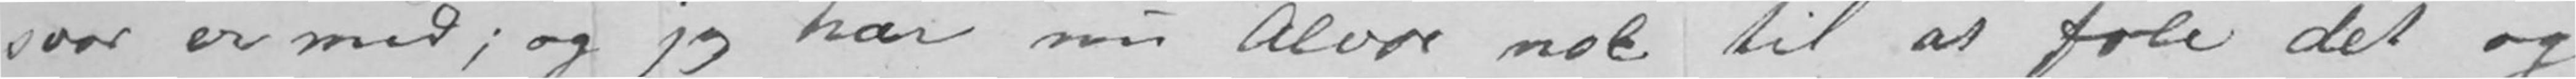svar er med; og jeg har nu Alvor nok til at føle det og
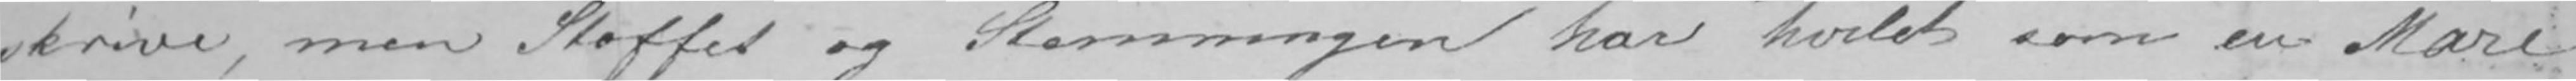skrive, men Stoffet og Stemningen har hvilet som en Mare
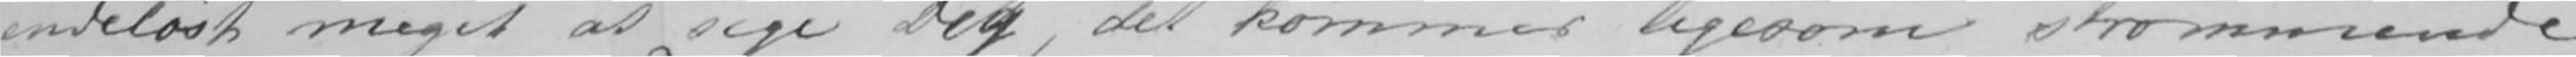endeløst meget at sige Dig, det kommer ligesom strømmende
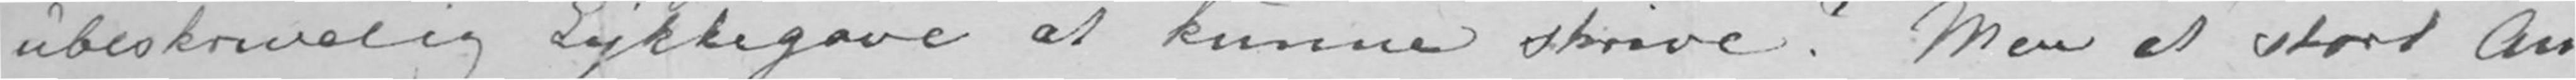ubeskrivelig Lykkegave at kunne skrive? Men et stort An-
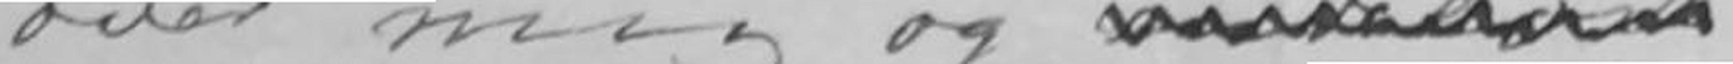over mig og
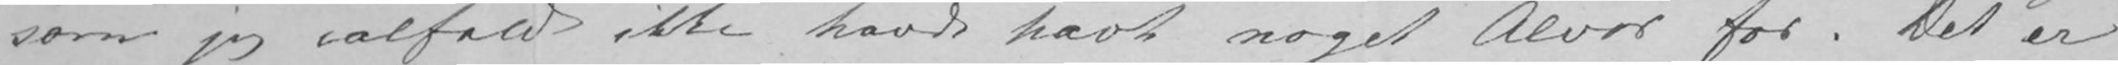som jeg ialfald ikke havde havt noget Alvor for. Det er
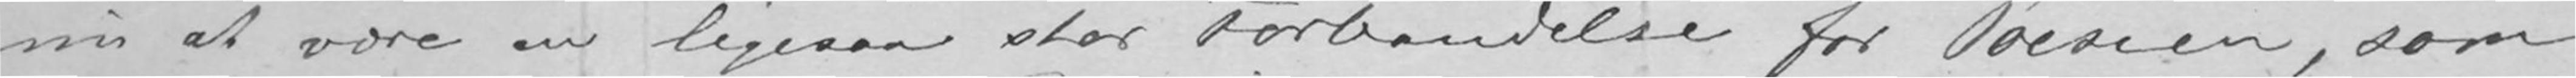nu at være en ligesaa stor Forbandelse for Poesien, som
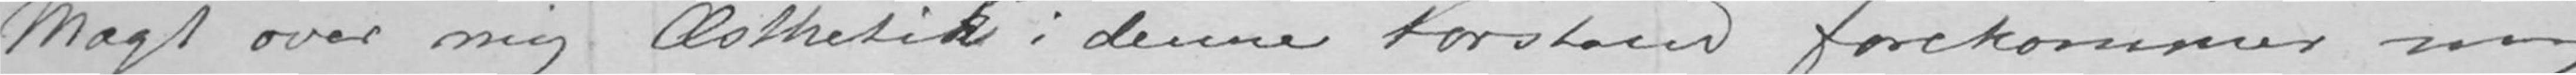Magt over mig Æsthetik i denne Forstand forekommer mig
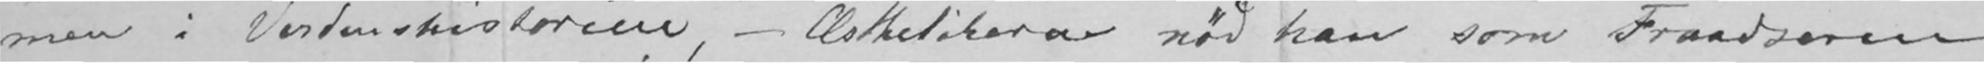men i Verdenshistorien,»- Æsthetikeren nød han som Fraadseren
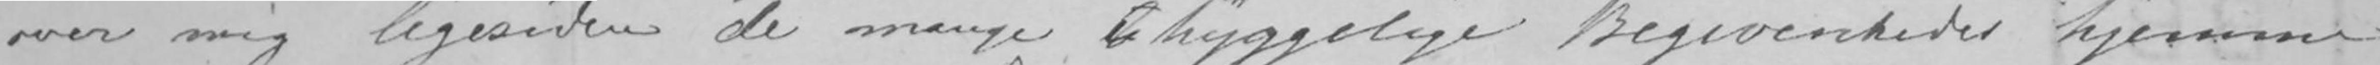over mig ligesiden de mange uhyggelige Begivenheder hjemme
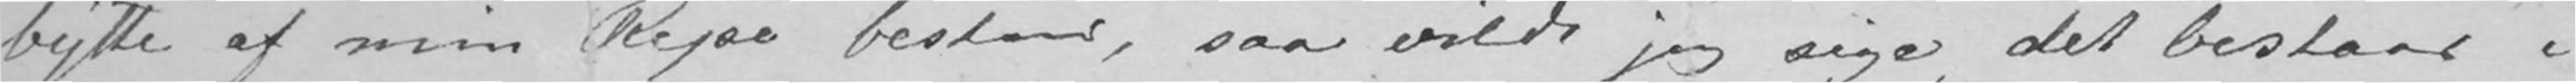bytte af min Rejse bestaar, saa vilde jeg sige, det bestaar i
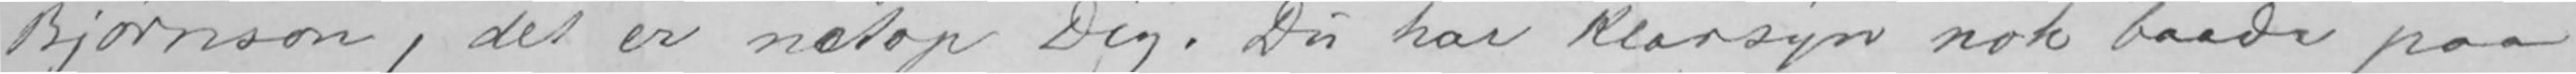Bjørnson, det er netop Dig. Du har Klarsyn nok baade paa
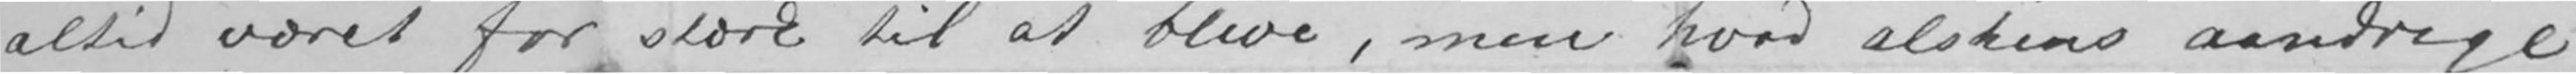altid været for stærk til at blive, men hvad alskens aandrige
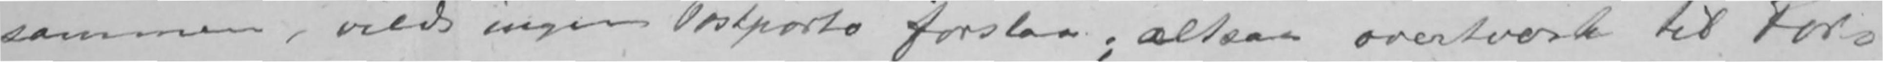sammen, vilde ingen Postporto forslaa; altsaa overtvært til For-
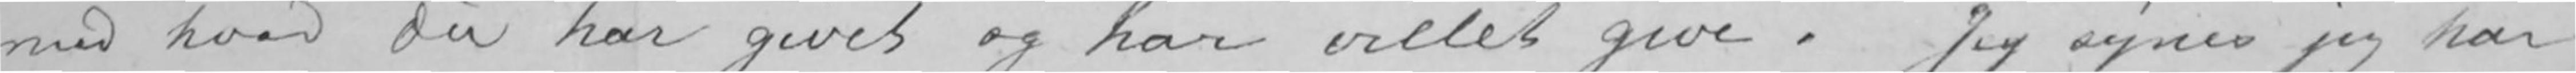med hvad Du har givet og har villet give. Jeg synes jeg har
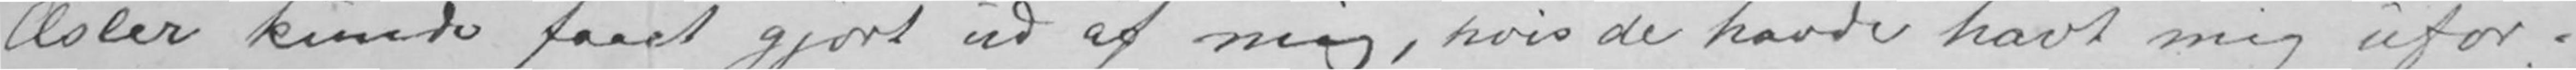Æsler kunde faaet gjort ud af mig, hvis de havde havt mig ufor-
